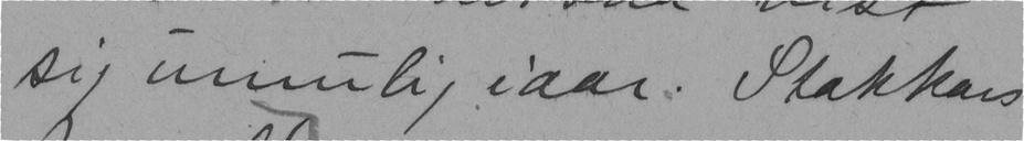sig umulig iaar. Stakkars
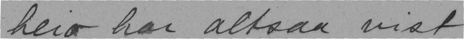heio har altsaa vist
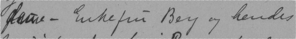- Enkefru Berg og hendes
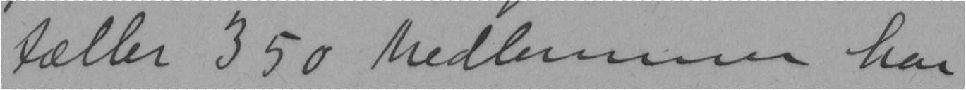tæller 350 Medlemmer har
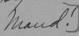Mand!
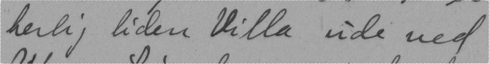herlig liden Villa ude ved
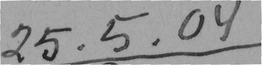25.5.04
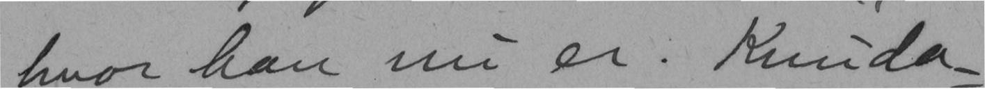hvor han nu er. Knuda-
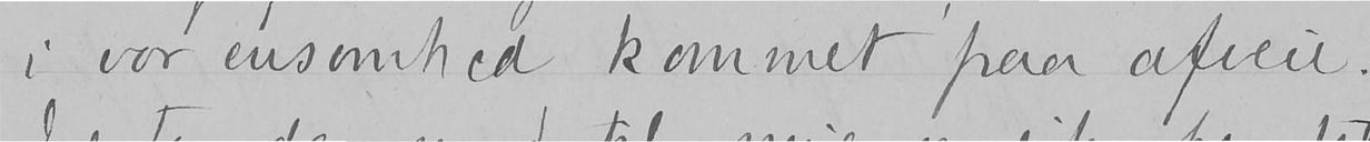i vor ensomhed kommet paa afveie.
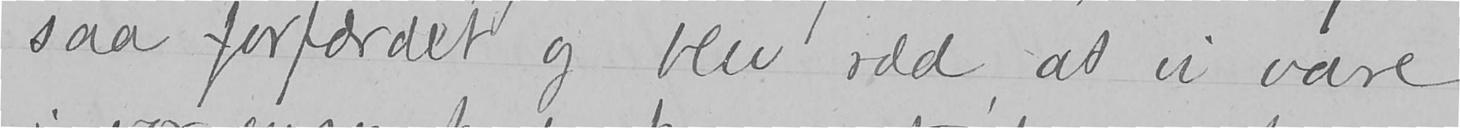saa forfærdet og blev ræd, at vi vare
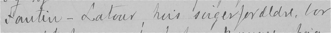Fantin-Latour, hvis svigerforældre bor
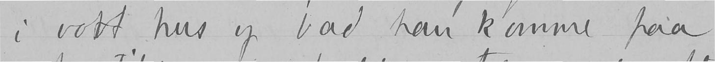i vort hus og bad han komme paa
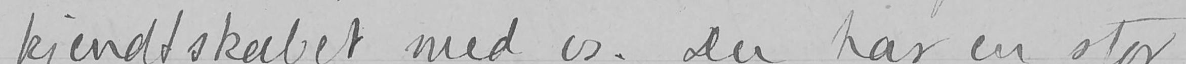kjendtskabet med os. Du har en stor
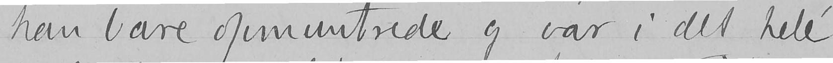han bare opmuntrede og var i det hele
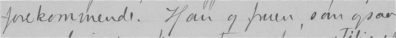forekommende. Han og fruen, som ogsaa
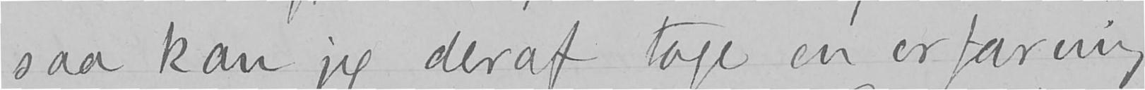saa kan jeg deraf tage en erfaring
